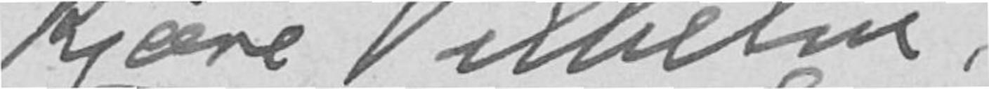Kjære Vilhelm,
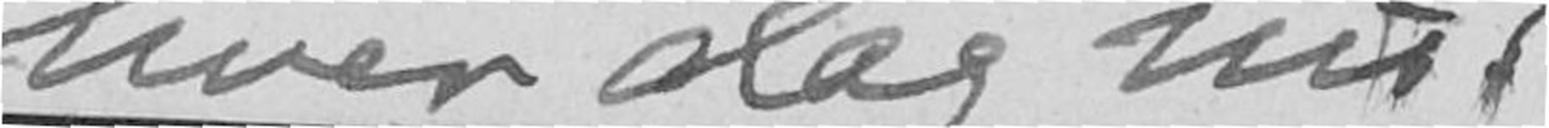hver dag nu!
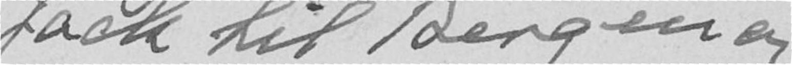Jack til Bergen og
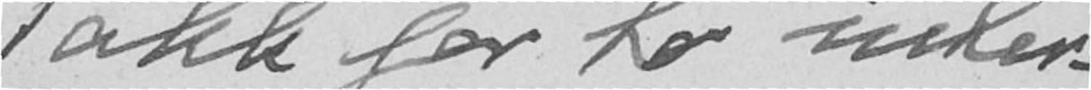Takk for to inter-
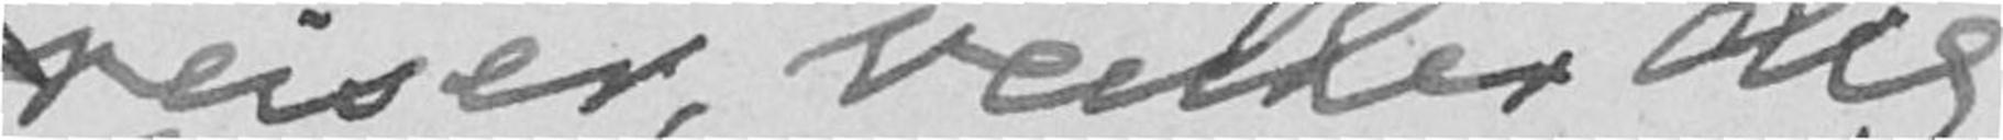reiser, venter dig
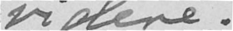videre.
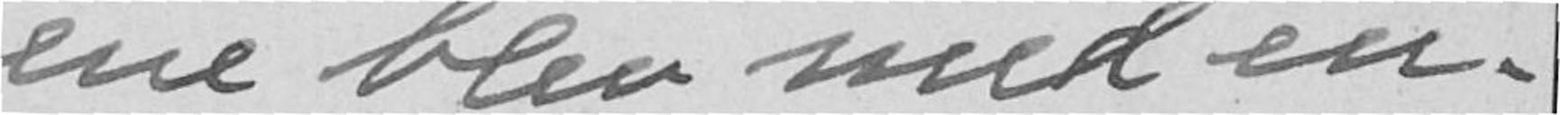ene blev med en-
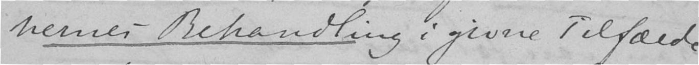nernes Behandling i givne Tilfælde
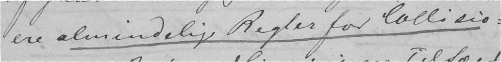ere almindelige Regler for Collisio-
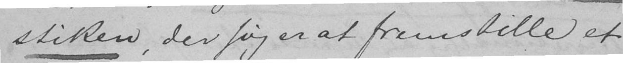stiken, der søger at fremstille et
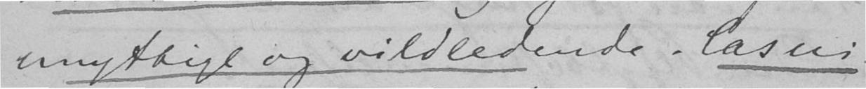unyttige og vildledende. Casui-
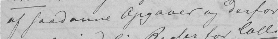af saadanne Opgaver og derfor
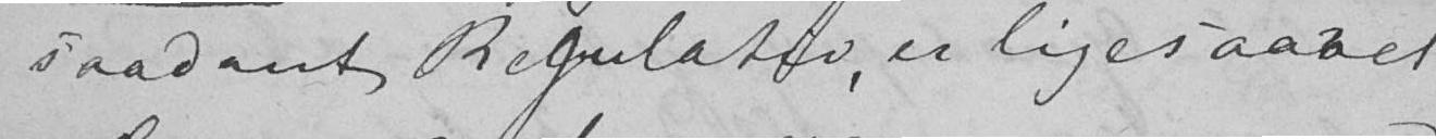saadant Regulativ, er ligesaavel
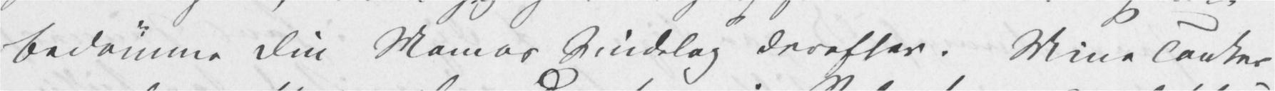bedømme Din Mamas Sindelag derefter. Mine Tanker
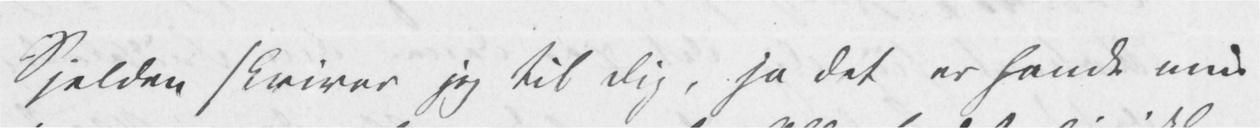Sjelden skriver jeg til Dig, ja det er sandt min
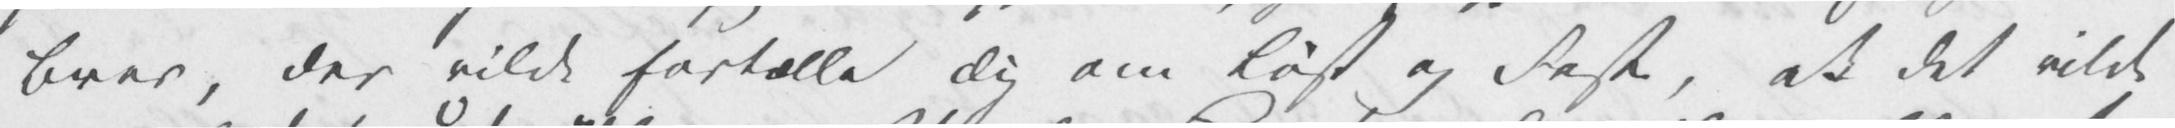Brev, der vilde fortælle Dig om Løst og Fast, ak det vilde
